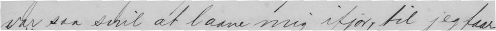var saa snil at laane mig ifjor, til jeg faar
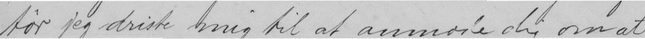tør jeg driste mig til at anmode dig om at
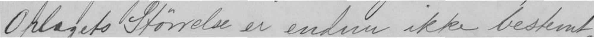Oplagets Størrelse er endnu ikke bestemt,
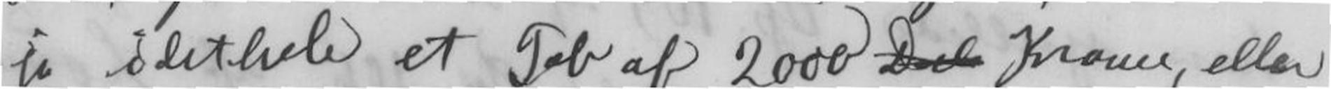jo idethele et Tab af 2000 Dal Kroner, eller
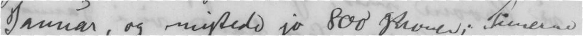Januar, og mistede jo 800 Kroner; Timerne
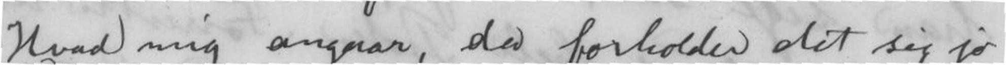Hvad mig angaar, da forholder det sig jo
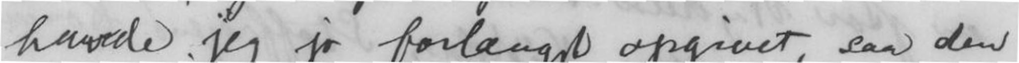havde jeg jo forlangt opgivet, saa den
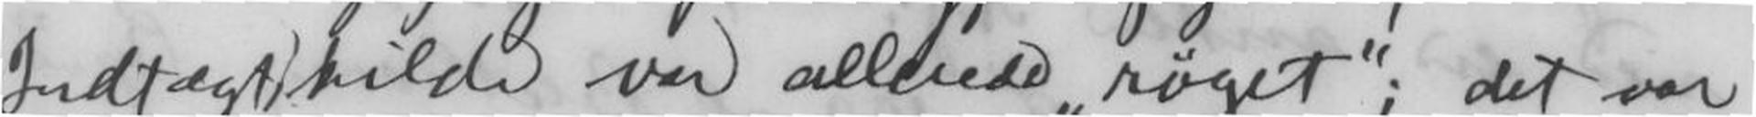Indtægtskilde var allerede røget; det var
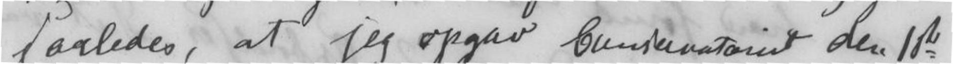saaledes, at jeg opgav Conservatoriet den 1ste
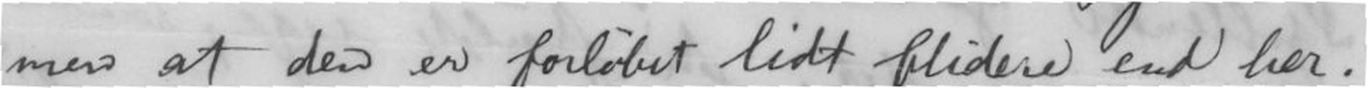men at den er forløbet lidt blidere end her!
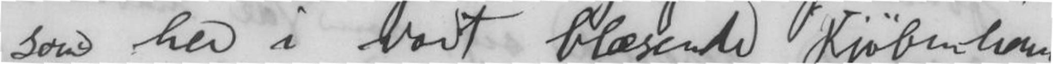som her i vort blæsende Kjøbenhavn,
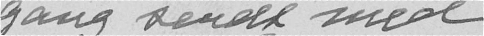gang sendt med
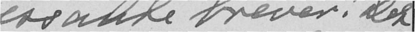essante brever! Det
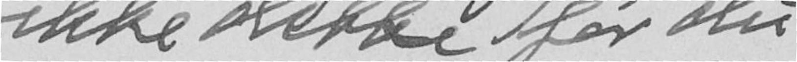ikke dette før du
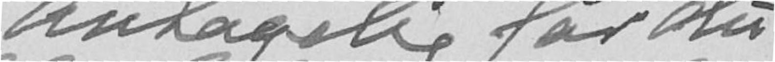Antagelig får du
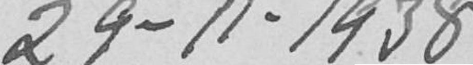29-11-1938
